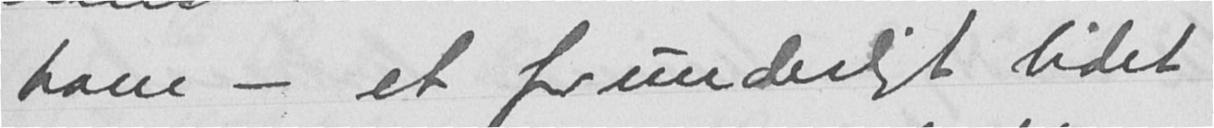ham - et forunderligt lidet
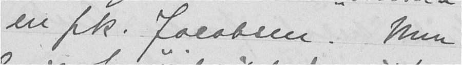en frk. Jacobsen. Men
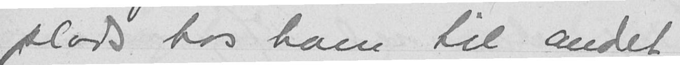plads hos ham til andet
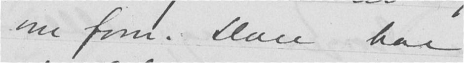om form. Han har
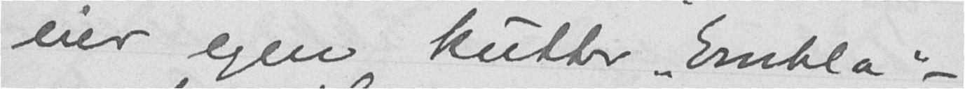eier egen kutter "Embla" -
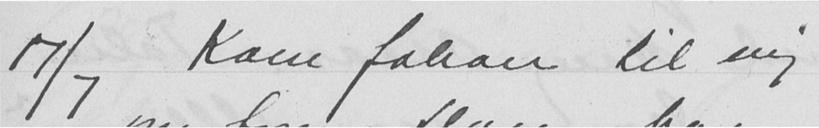17/3 Kom Johan til mig
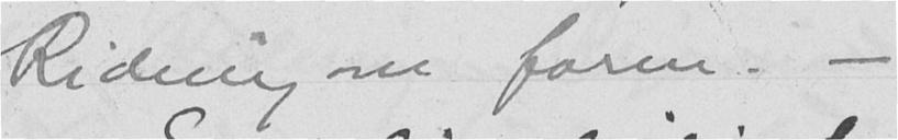Ridning om form. -
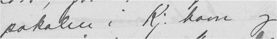pokalen i Kj. havn og
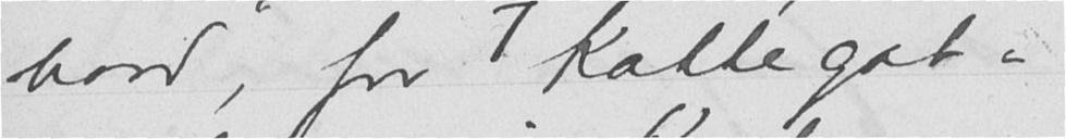bord, for Kattegat-
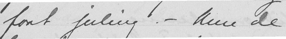faat juling. - Men de
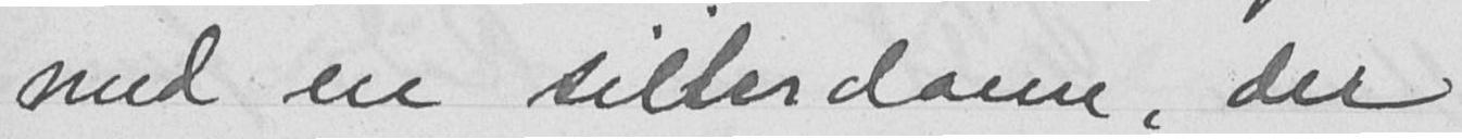med en seilerdame, der
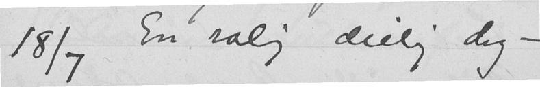18/7 En rolig deilig dag -
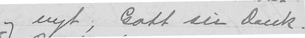og nyt, Gott sei Dank.
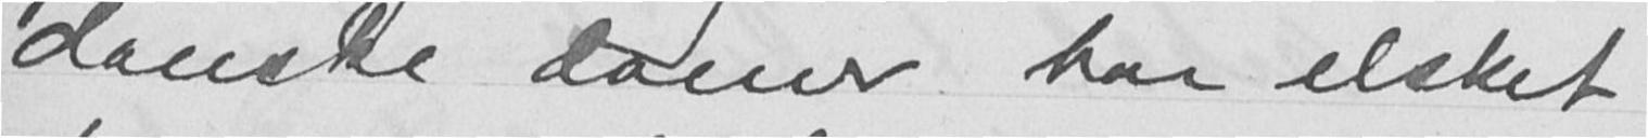danske damer har elsket
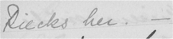Riecks her. -
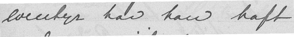eventyr har han haft
